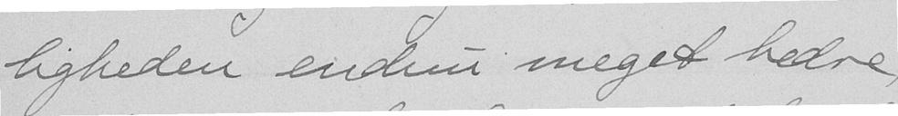ligheden endnu meget bedre
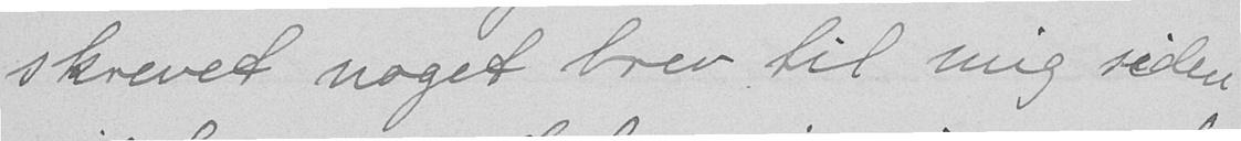skrevet noget brev til mig siden
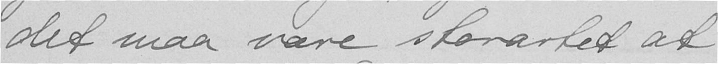det maa være storartet at
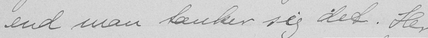end man tænker sig det. Har
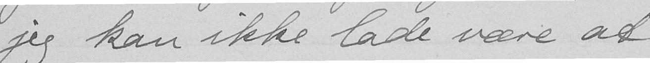jeg kan ikke lade være at
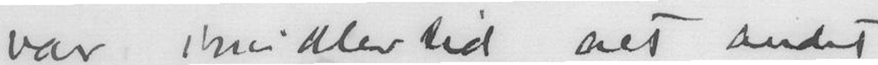var imidlertid alt andet
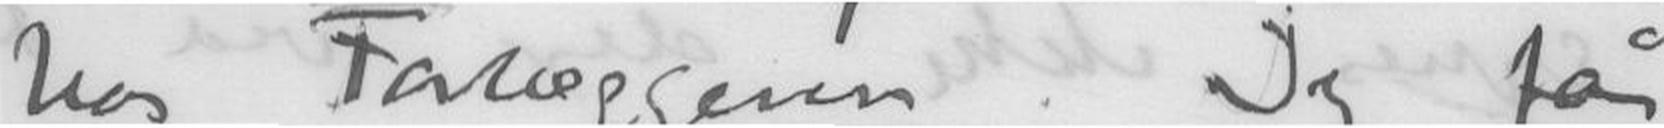hos Forlæggeren. Jeg får
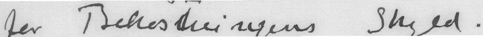for Bekostningens Skyld.
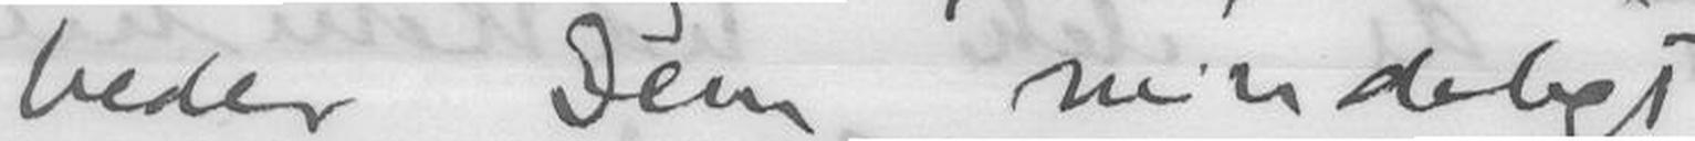beder Dem uendeligt
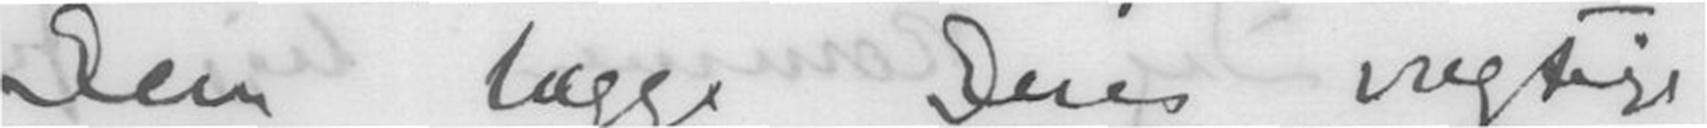Dem lægge Deres vægtige
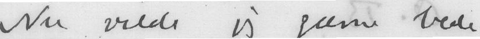Nu vilde jeg gærne bede
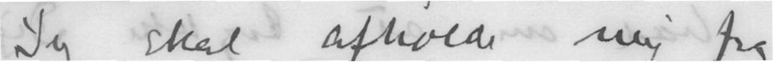Jeg skal afholde mig fra
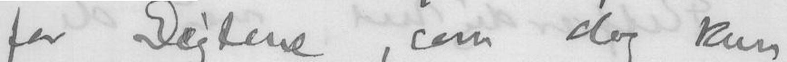for Digtene, som dog kun
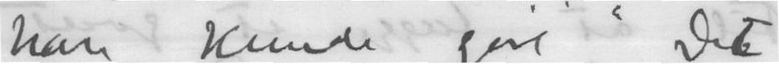han kunde gøre Det
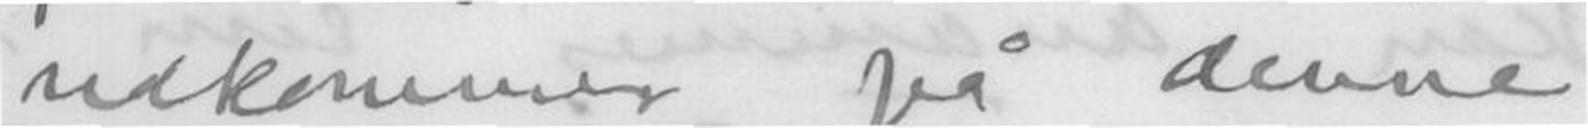udkommer på denne
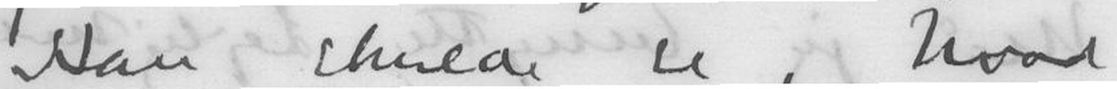Han skulde se, hvad
